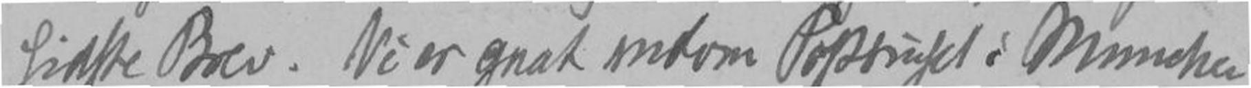sidste Brev. Vi er gaaet indom Posthuset i Munchen
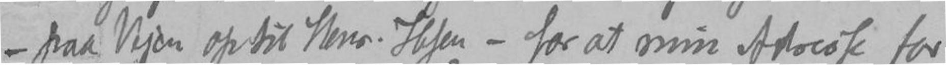-paa Vejen op til Henr. Ibsen - for at min Adresse for
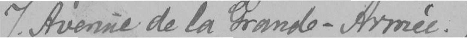"I. Avenue de la Grande - Armée. 7"
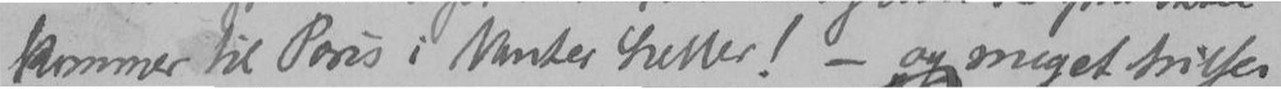kommer til Paris i Vinter heller! - og meget hilses
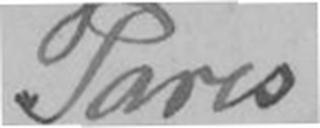Paris
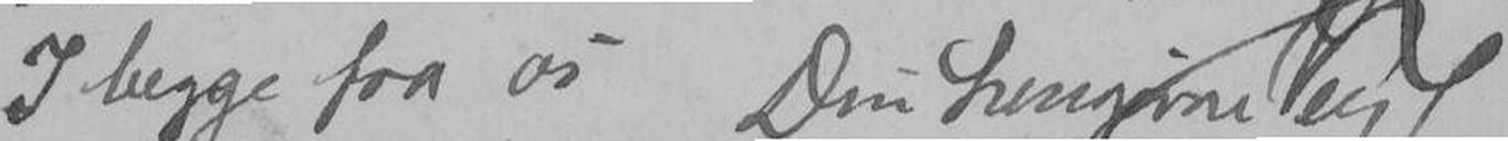I begge fra os Din hengivne Ven
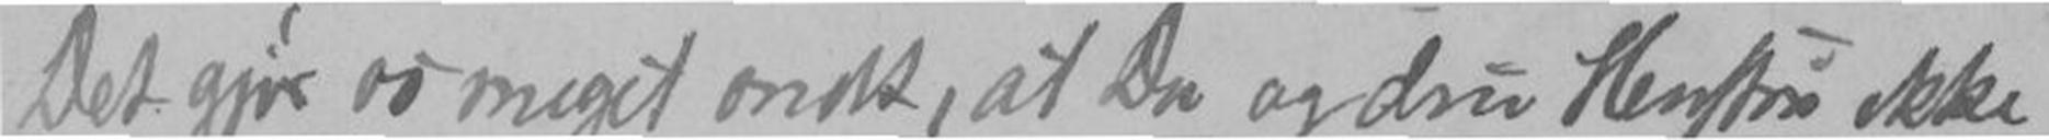Det gjør os meget ondt, at Du og din Hustru ikke
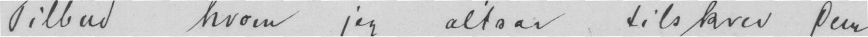Tilbud hvem jeg altsaa tilskrev Den
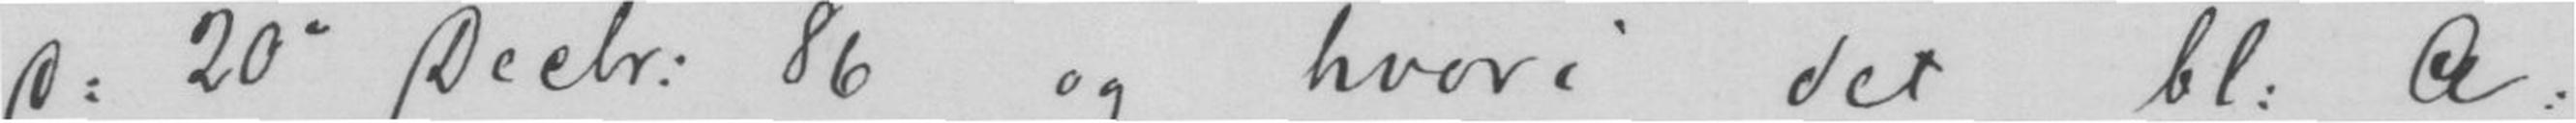p= 20 Decbr. 86 og hvori det bl. A.
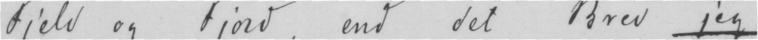Fjeld og Fjord, end det Brev jeg
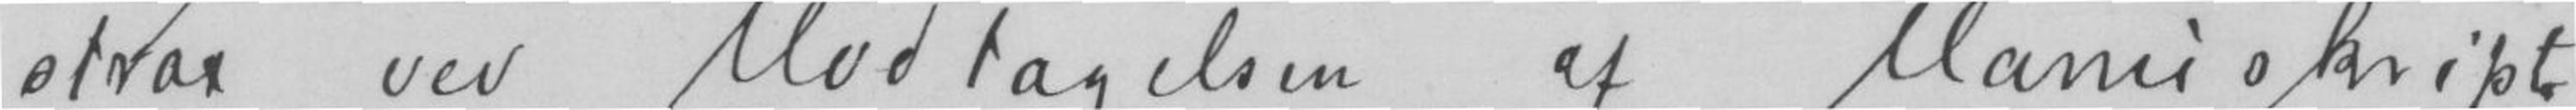strax ved Modtagelsen af Manuskript-
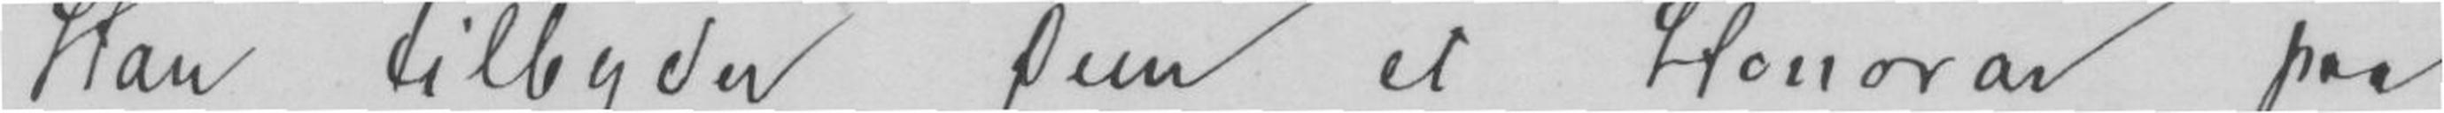Han tilbyder dem et Honorar paa
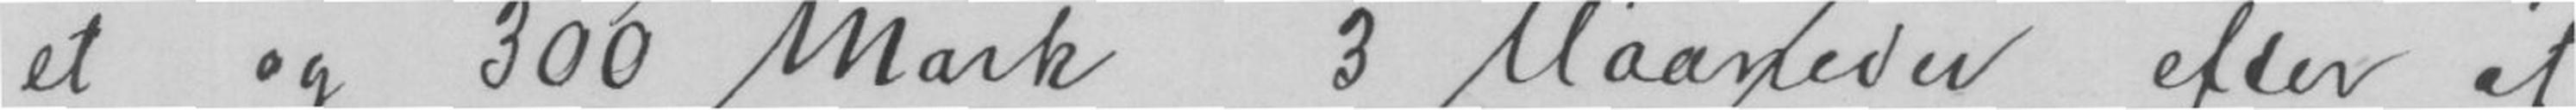et og 300 Mark 3 Maaneder efter at
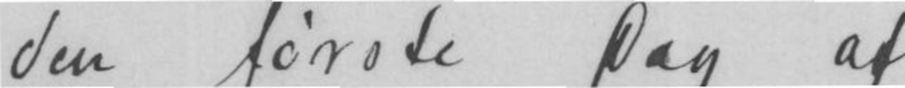den første Dag af.
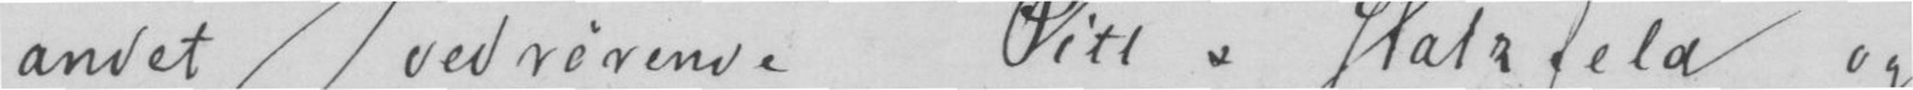andet vedrørende Pitt & Hatzfeld og
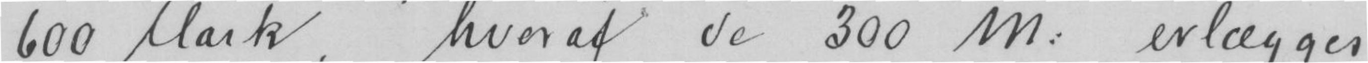600 Mark, hvoraf de 300 M: erlægges
